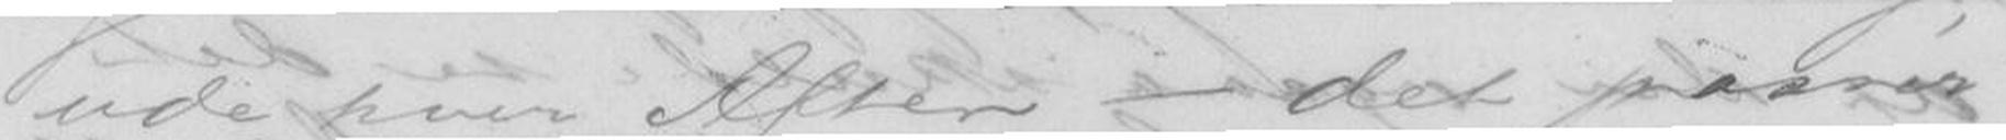ude hver aften- det passer
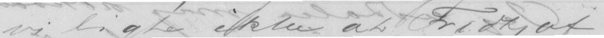vi ligte ikke at Fridtjof
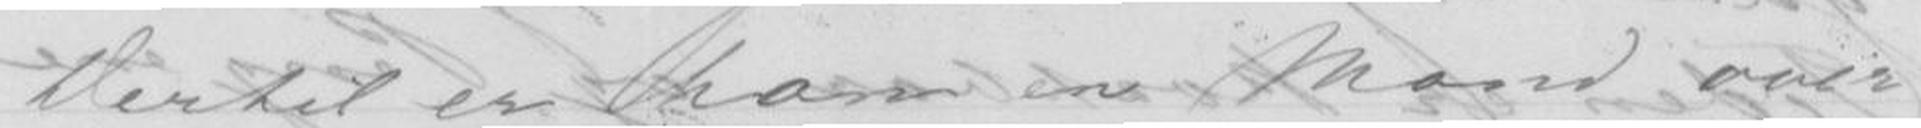Dertil er han en Mand over
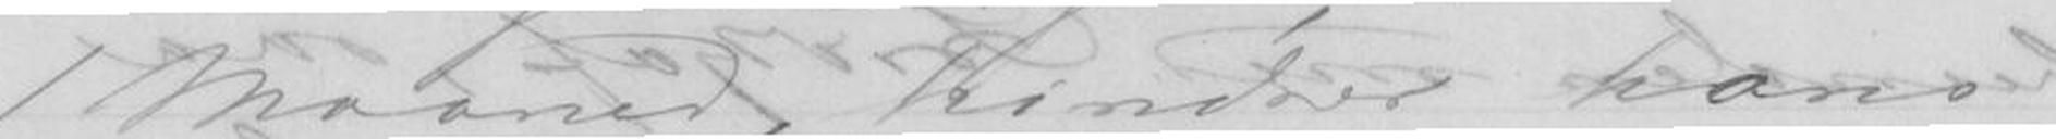1 Maaned, hindrer hans
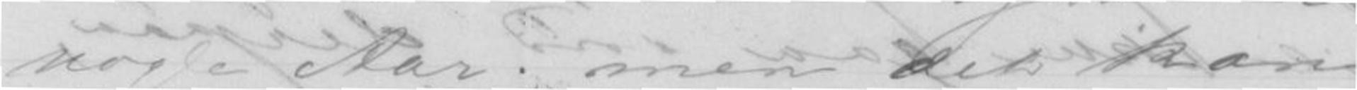nogle Aar; men det kan
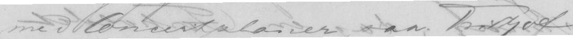med Concertplaner, saa Fridtjof
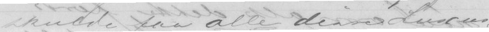skulde faa alle disse Luxus-
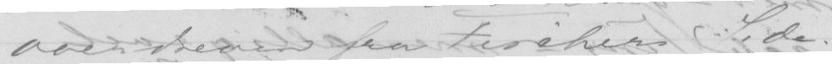overdreven fra Fischers Side.
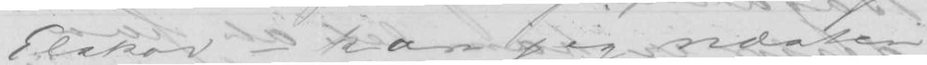Elskov - kan jeg næsten
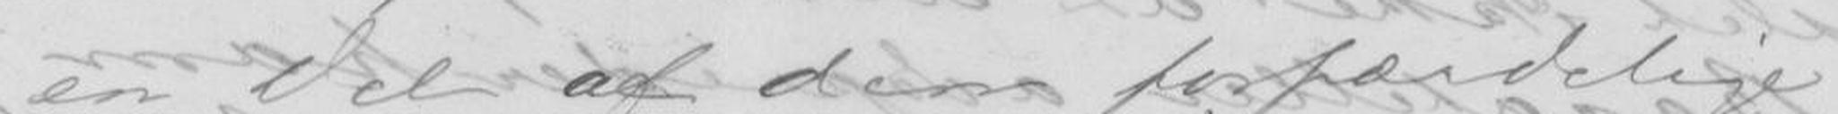en Del af den forfærdelige
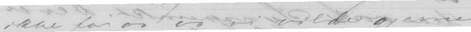ikke for os og vi vilde gjerne
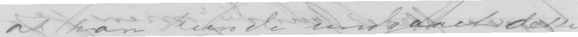at han kunde undgaaet dette
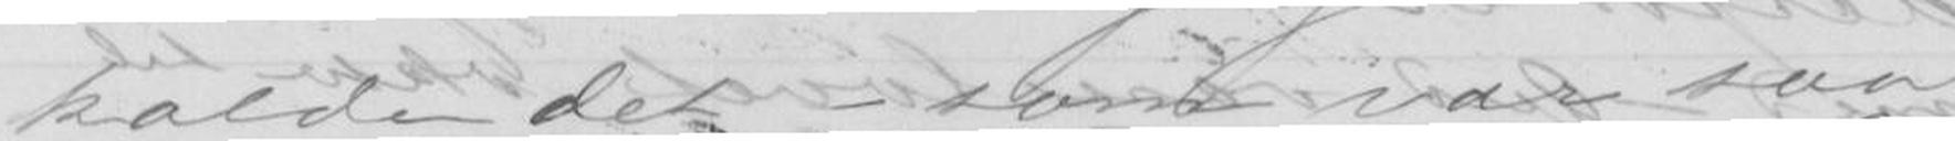kalde det - som var saa
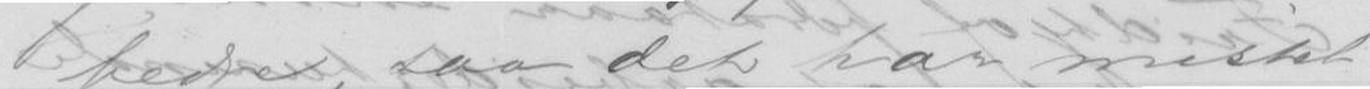bedre, saa det har mistet
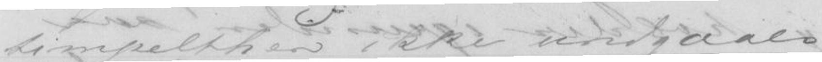simpelthen ikke undgaaes,
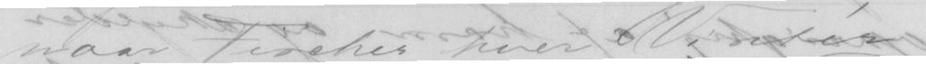naar Fischer hver Vinter
